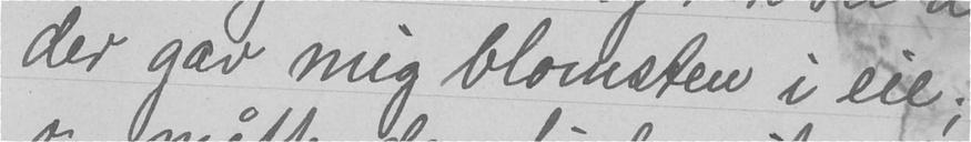der gav mig blomsten i eie;
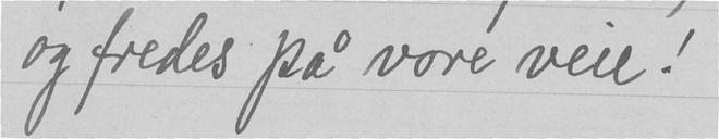og fredes på vore veie!
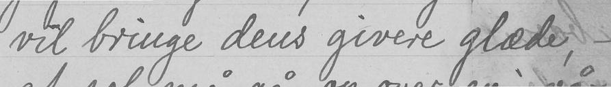vil bringe dens givere glæde, -
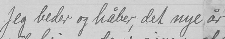Jeg beder og håber, det nye år
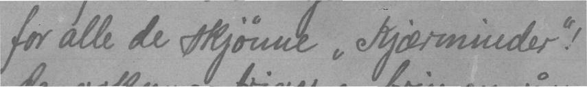for alle de skjønne "kjærminder"!
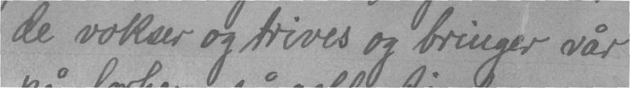de vokser og trives og bringer vår
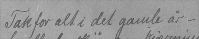Tak for alt i det gamle år -
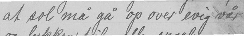at sol må gå op over evig vår
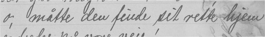o, måtte den finde sit rette hjem
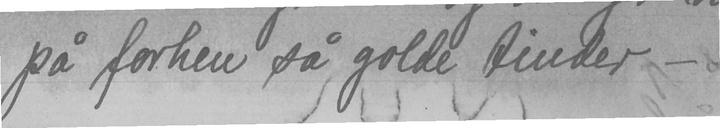på forhen så golde tinder -
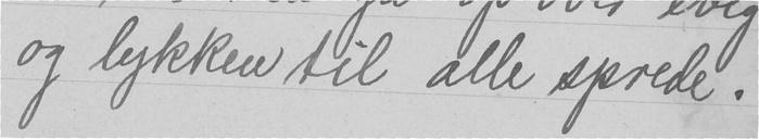og lykken til alle sprede.
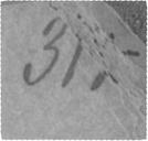319.
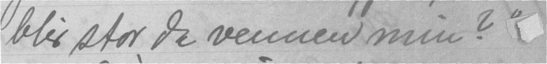blir stor da vennen min?"
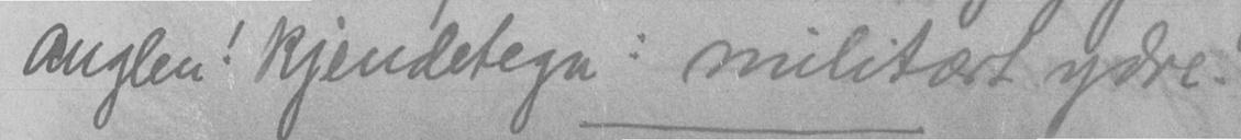anglen! kjendetegn: militært ydre.
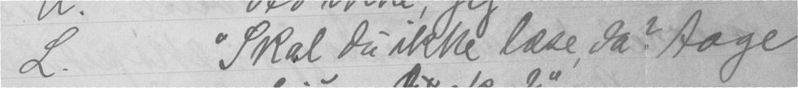L. "Skal du ikke læse, da? tage
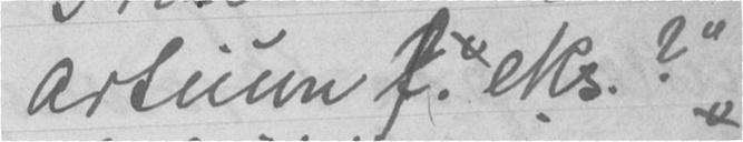artium f.eks.?"
